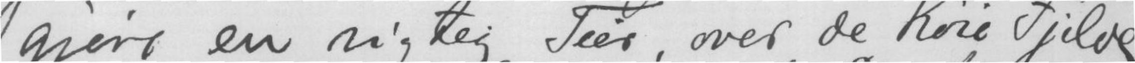gjøre en rigtig Tur, over de høie Fjelde.
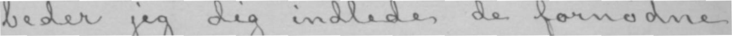beder jeg dig indlede de fornødne
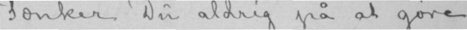Tænker Du aldrig på at gøre
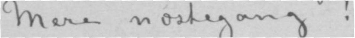Mere næstegang!
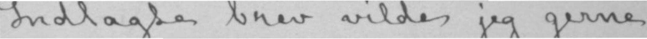Indlagte brev vilde jeg gerne
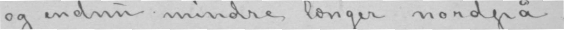og endnu mindre længer nordpå.
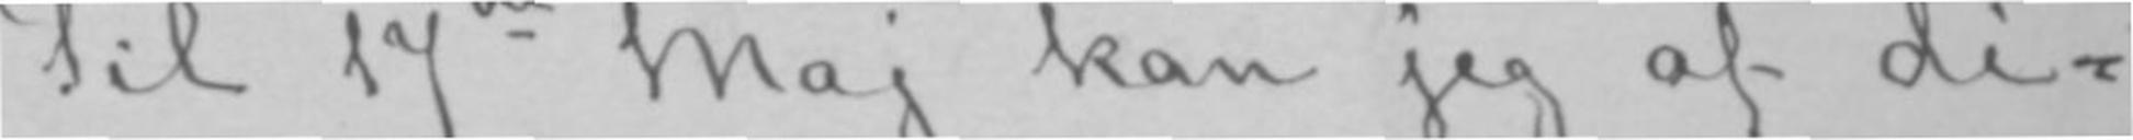Til 17de Maj kan jeg af di-
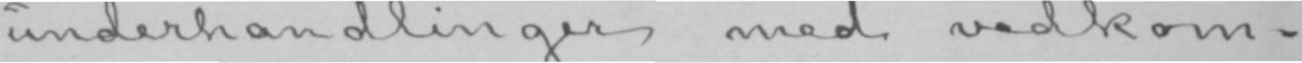underhandlinger med vedkom-
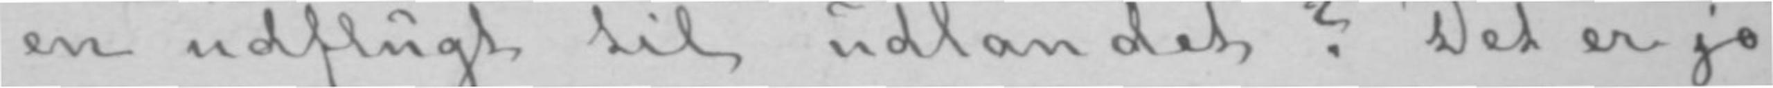en udflugt til udlandet? Det er jo
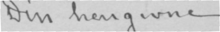Din hengivne
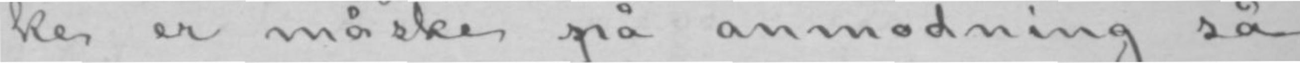ke er måske på anmodning så
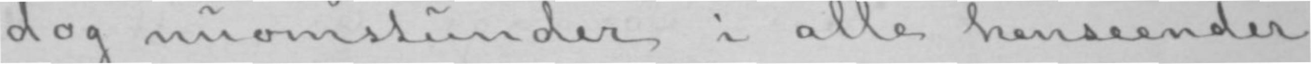dog nuomstunder i alle henseender
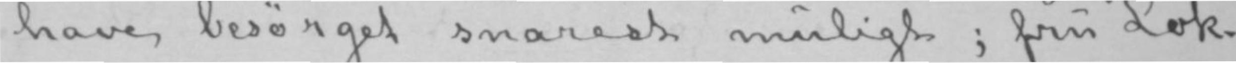have besørget snarest muligt; fru Løk-
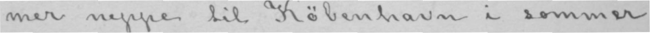mer neppe til København i sommer,
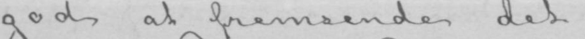god at fremsende det.
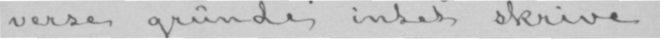verse grunde intet skrive.
